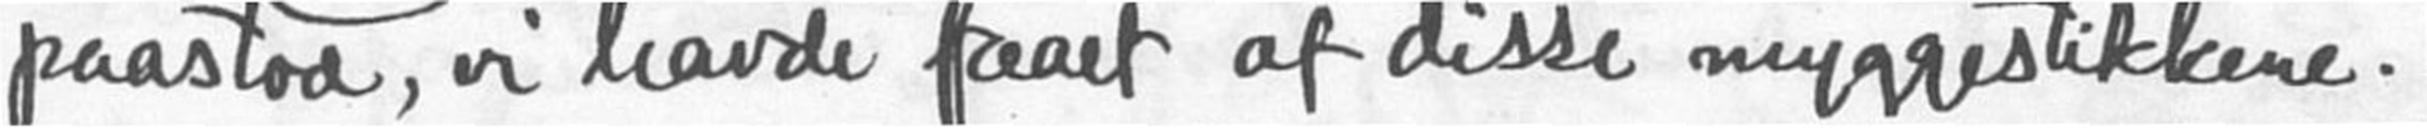paastod, vi havde faaet af disse myggestikkene. -
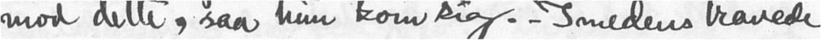mod dette, saa hun kom sig. - Imedens travede
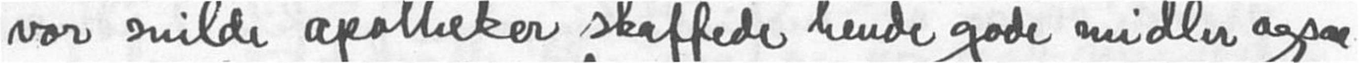vor snilde apotheker skaffede hende gode midler ogsaa
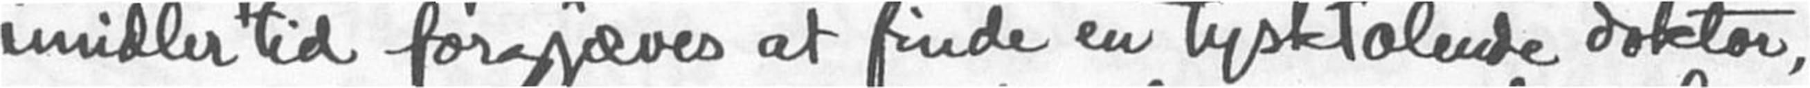imidlertid forgjæves at finde en tysktalende doktor,-
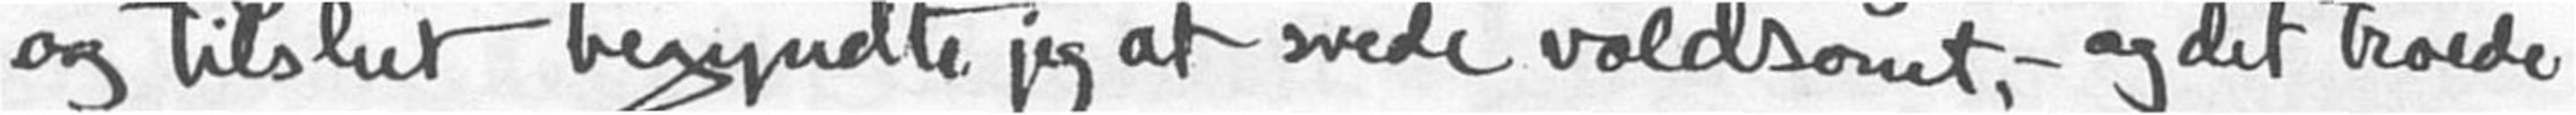og tilslut begyndte jeg at svede voldsomt, - og det troede
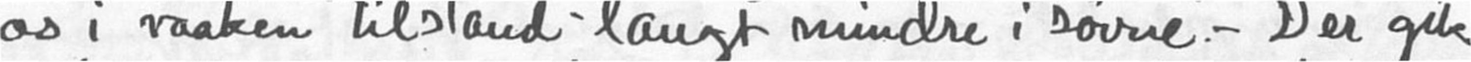os i vaaken tilstand - langt mindre i søvne. - Der gik
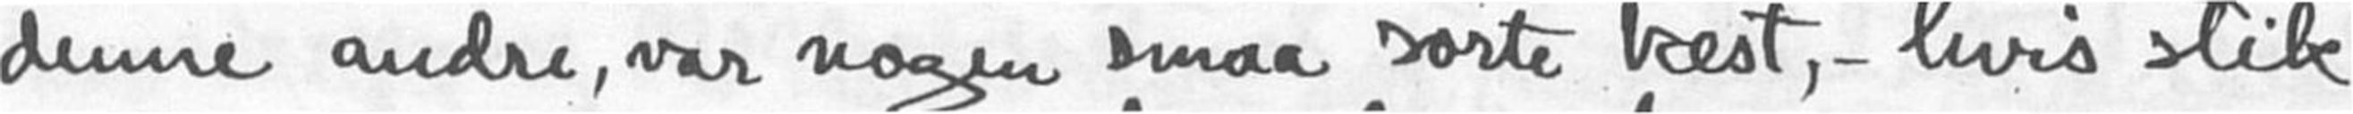denne andre, var nogen smaa sorte best, - hvis stik
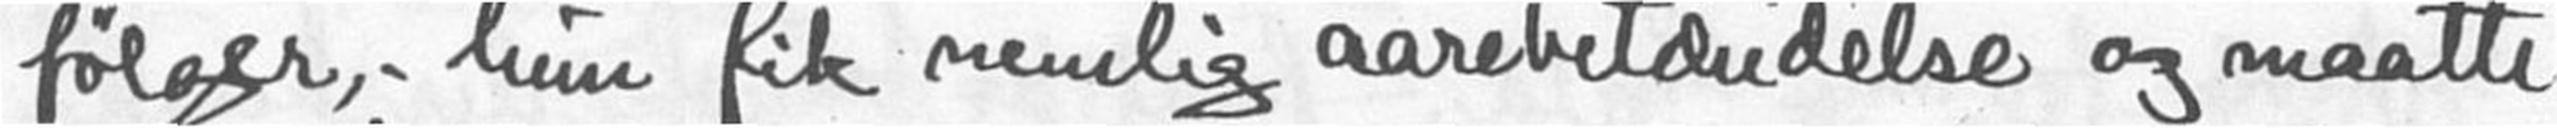følger, - hun fik nemlig aarebetændelse og maatte
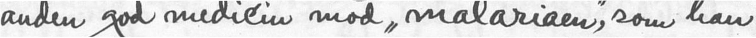anden god medicin mod "malariaen" som han
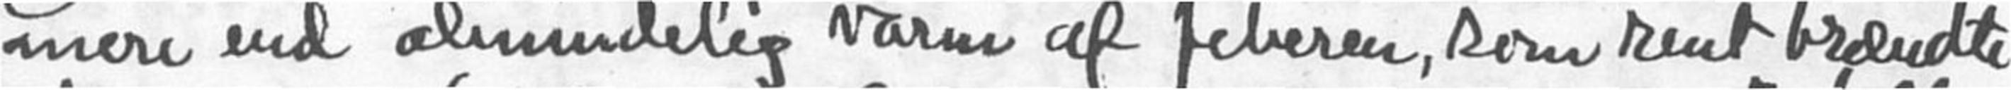mere end almindelig varm af feberen, som rent brændte
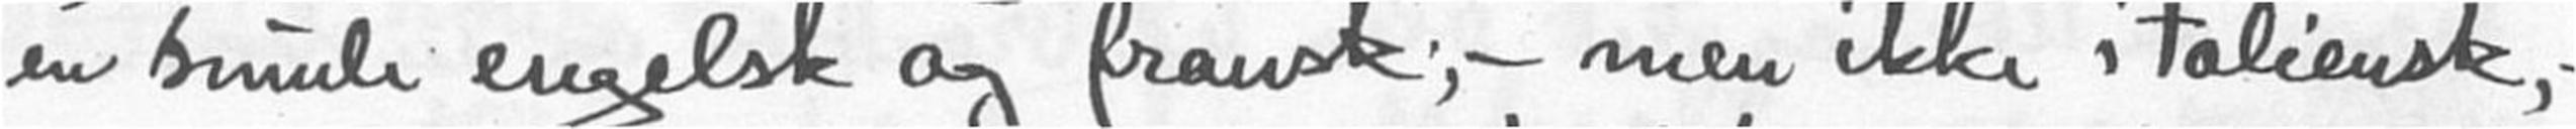en smule engelsk og fransk; - men ikke italiensk,-
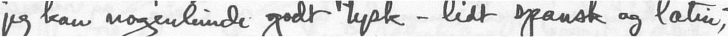jeg kan nogenlunde godt tysk - lidt spansk og latin,
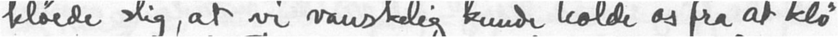kløede slig, at vi vanskelig kunde holde os fra at klø
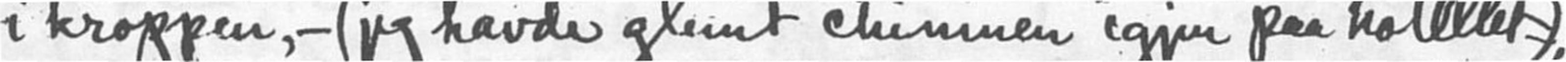i kroppen, - (jeg havde glemt chininen igjen paa hotellet)
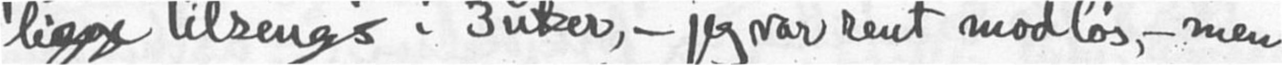ligge tilsengs i 3 uker, - jeg var rent modløs, - men
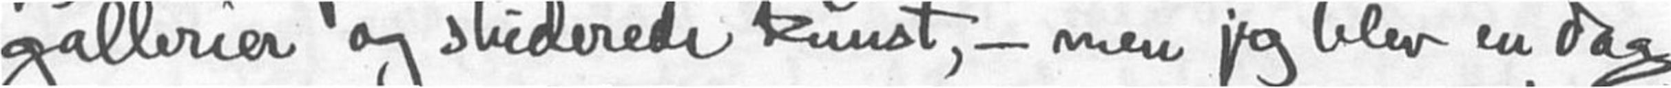gallerier og studerede kunst, - men jeg blev en dag
